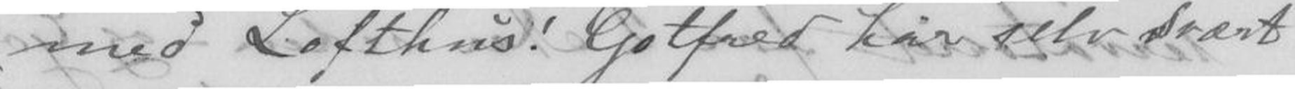med Lofthus! Gotfred har selv svært
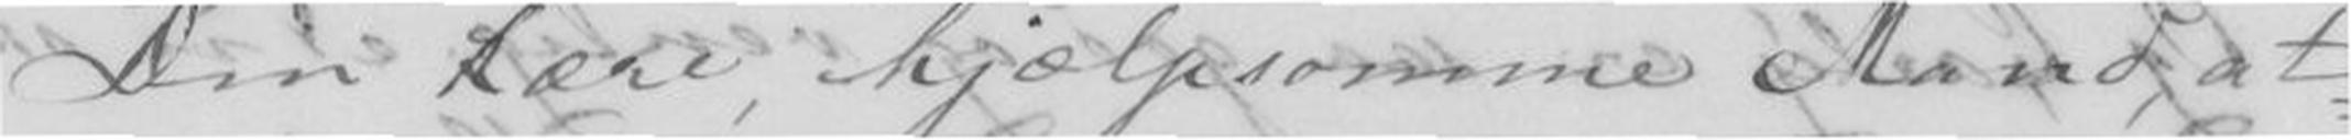Din kære hjælpsomme Mand, at-
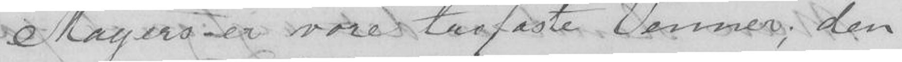Mayers er vore trofaste Venner; den
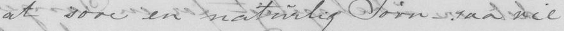at sove en naturlig Søvn - saa vil
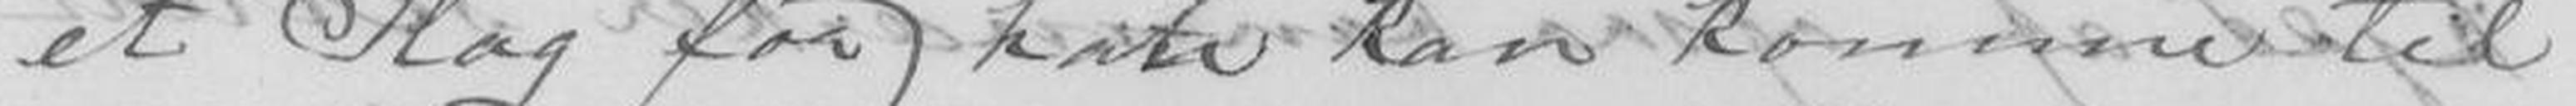et Slag for han kan komme til
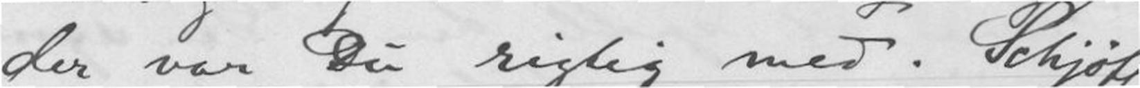der var Du rigtig med. Schjøtt
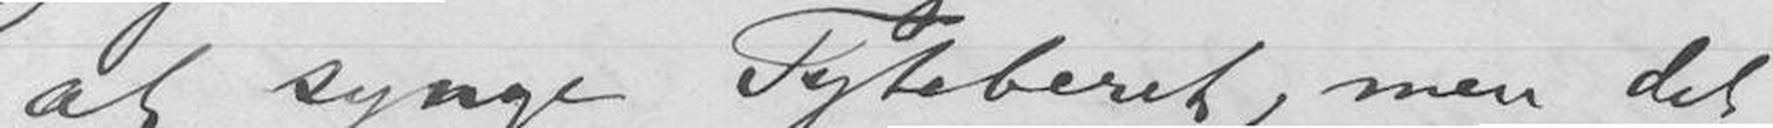at synge Tyteberet, men det
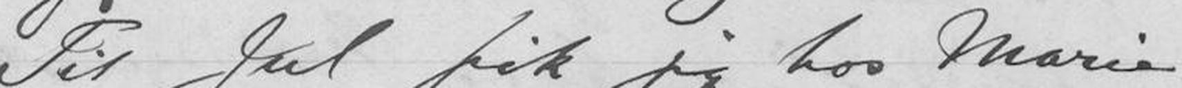Til Jul fik jeg hos Marie
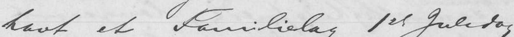havt et Familielag 1ste Juledag
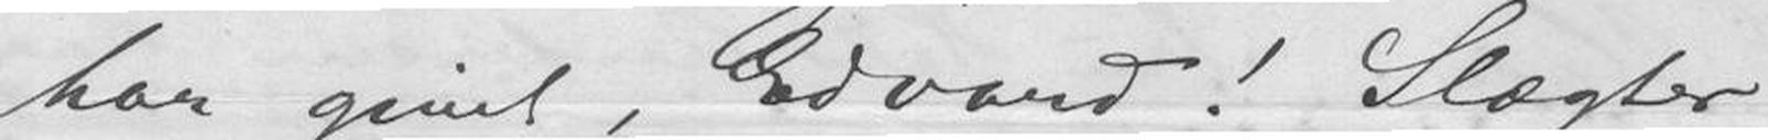har givet, Edvard! Slægter
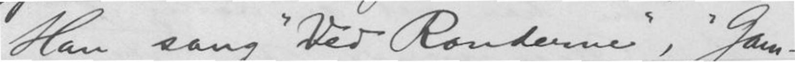Han sang "Ved Ronderne", "Gam-
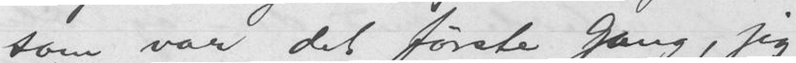som var det første Gang, jeg
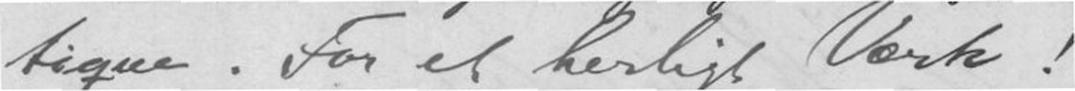tique. For et herligt Værk!
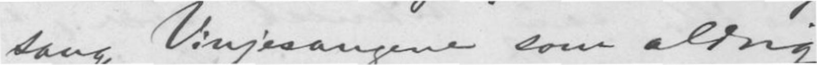sang Vinjesangene som aldrig
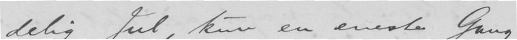delig Jul, kun en eneste Gang
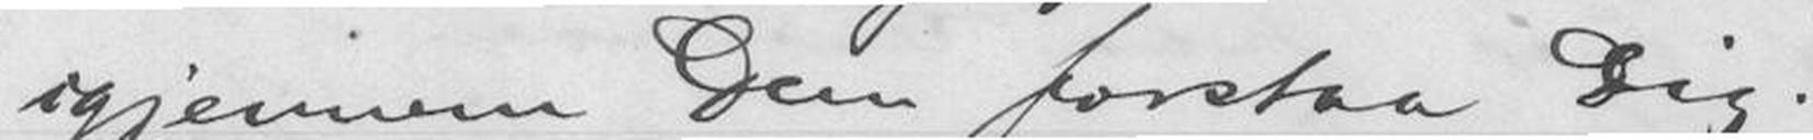igjennem Dem forstaa Dig.
